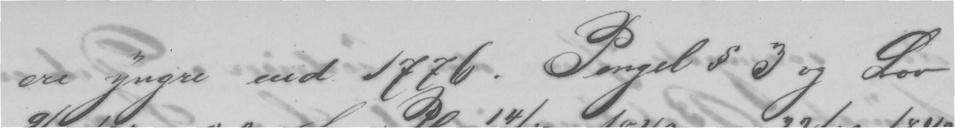ere yngre end 1776. Pengel §3 og Lov
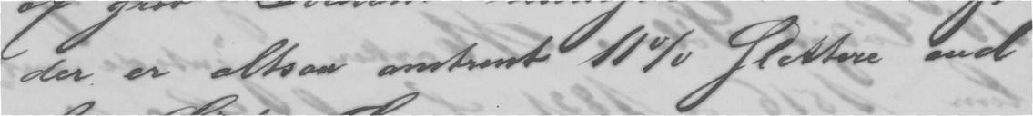der er altsaa omtrent 11% slettere end
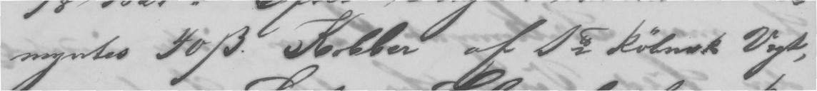myntes 40ẞ . Kobber af 1 daler kølnsk Vægt,
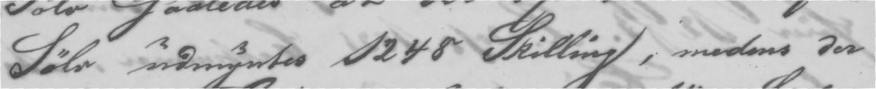Sølv udmyntes 1248 Skilling, medens der
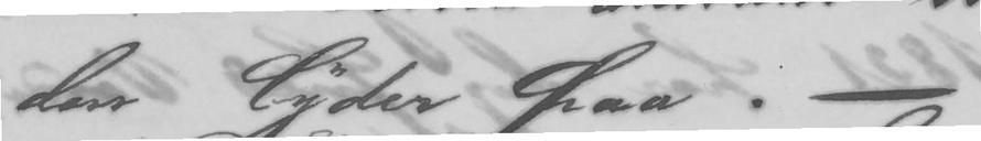der lyder paa. -
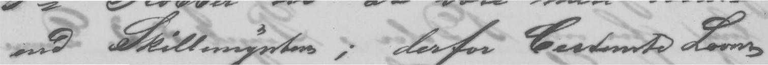end Skillmynter; derfor bestemte Loven
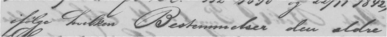ifølge hvilken Bestemmelser den ældre
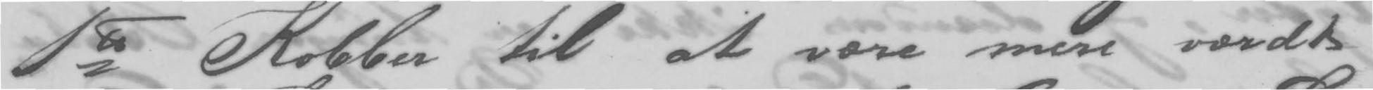1 daler Kobber til at være mere værdt
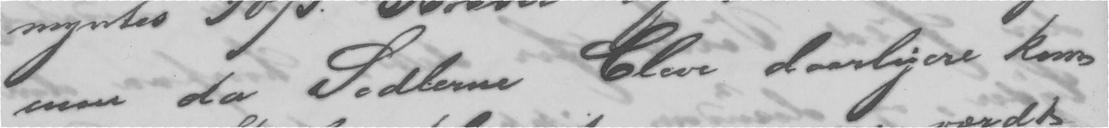men da Sedlene bleve daarligere kom
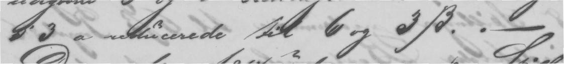§3 a reducerede til 6 og 3 ẞ. -
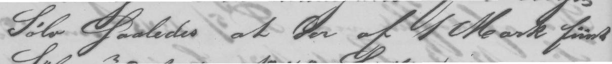Sølv Saaledes at den af 1 Mark fiint
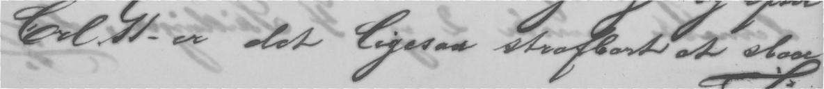Erl 11- er det ligesaa strafbart at slaae
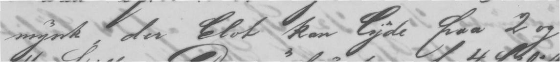mynt, der blot kan lyde paa 2 og
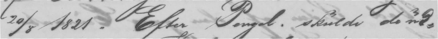20/8 1821. Efter Pengel. skulde de ud-
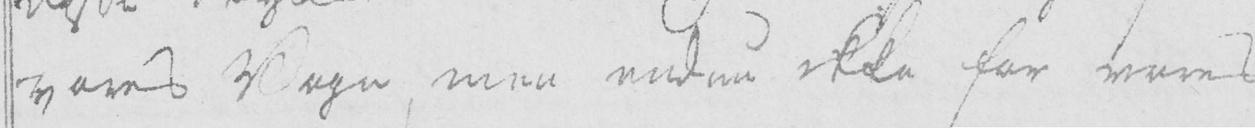vores Vogn, men endnu ikke for vores
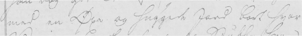med en Øxe og huggede Jord bort hvor
